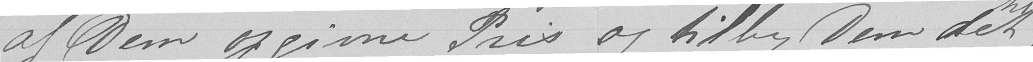af Dem opgivne Pris og tilby Dem det
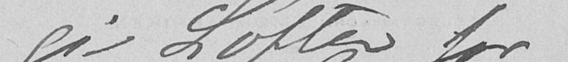gi Løfter for
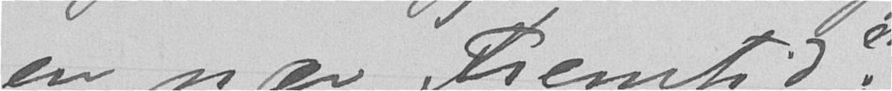en nær fremtid;
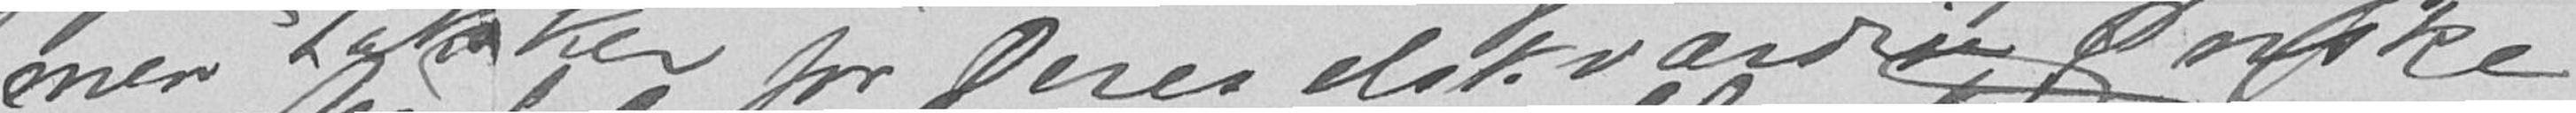men takker for Deres elskværdige Ønske.
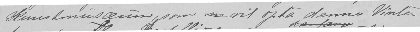Kunstmusæum, som  vil opta denne Vinter
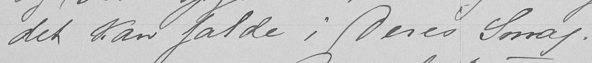det kan falde i Deres Smag.
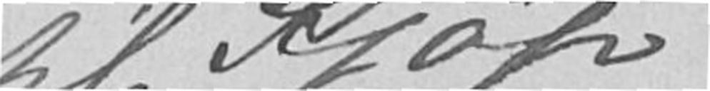til Kjøp
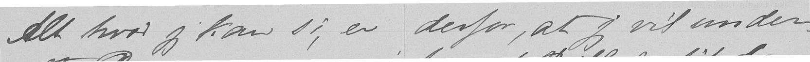Alt hvad jeg kan si, er derfor, at jeg vil under-
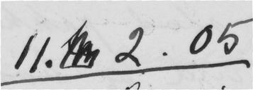11. 2.05
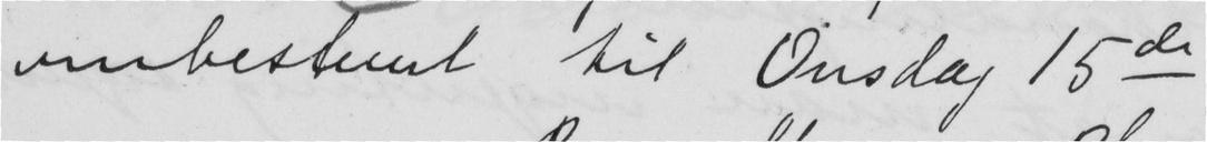ombestemt til Onsdag 15de.
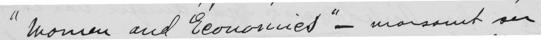"Women and Economies" - morsomt ser
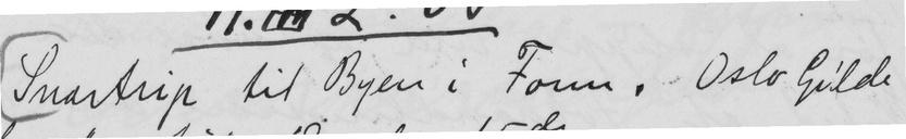Snartrip til Byen i Form. Oslo Gilde
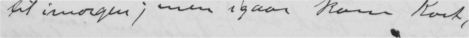til imorgen; men igaar kom Kort,
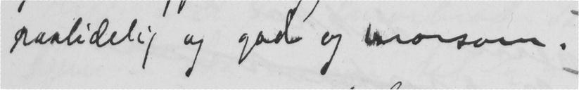paalidelig og god og morsom.
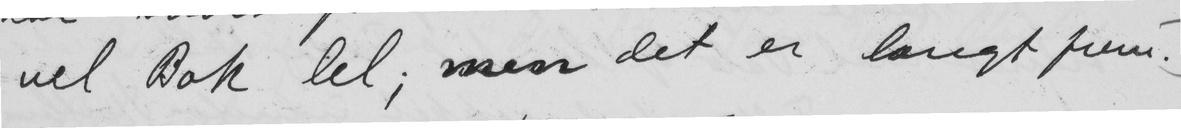vel Bok lel; men det er langt frem.
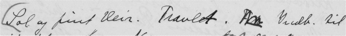Sol og fint Veir. Travlet.  Indb. til
